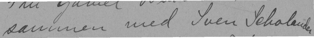sammen med Sven Scholander
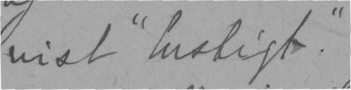vist "hustigt".
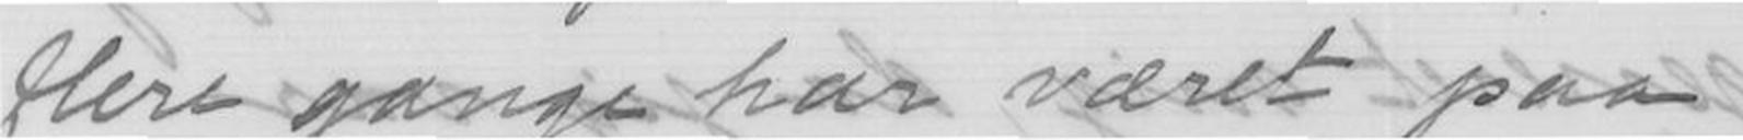flere ganger har været paa
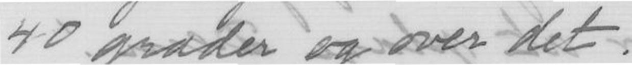40 grader og over det.
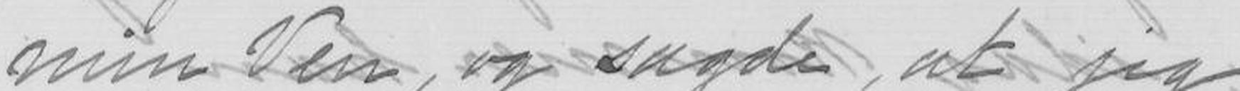min Ven, og sagde at jeg
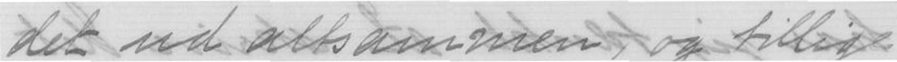det ud altsammen, og tidlige
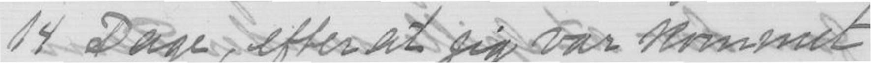14 Dage, efter at jeg var kommet
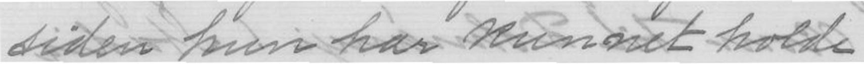siden hun har kunnet holde
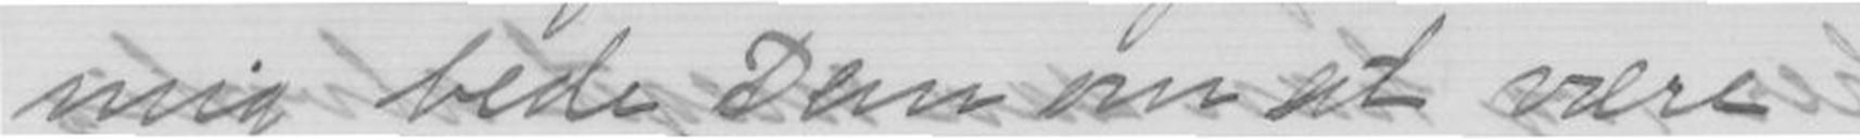mig bede Dem om at være
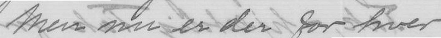Men nu er der for hver
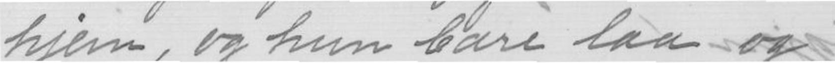hjem, og hun bare laa og
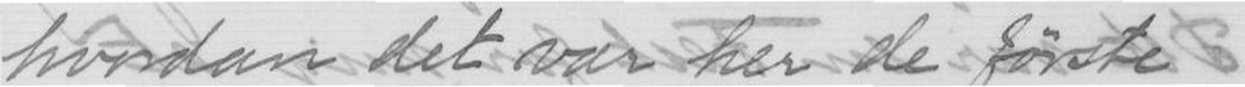hvordan det var her de første
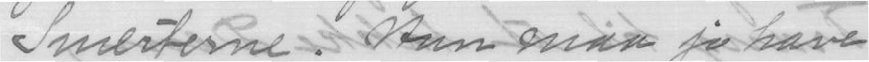Smertene. Hun maa jo have
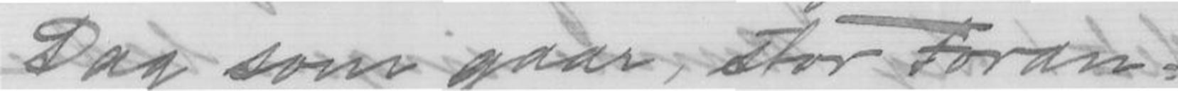Dag som gaar, Stor Foran-
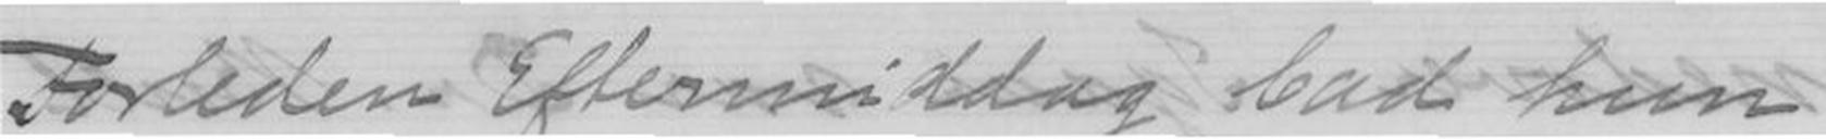Forleden Eftermiddag bad hun
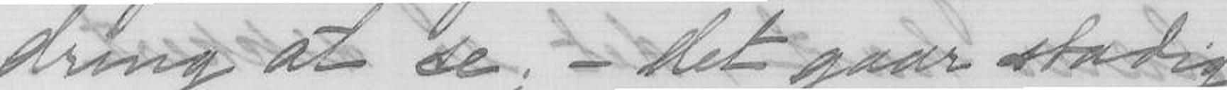dring at be; - det gaar stadig
